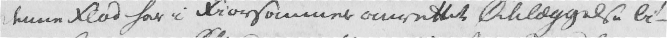denne Flod har i Fiorsommer anrettet Ødelæggelse li-
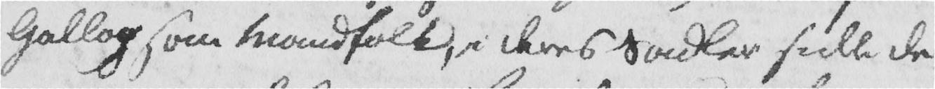Gallop som Mandfolk, i deres Sadler sidde de
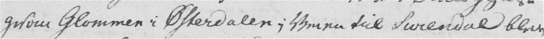gesom Glommen i Østerdalen; Veien til Surendal blev
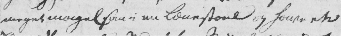meget magel som i en Lænestoel og have et
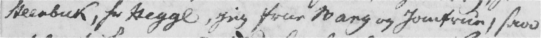Steenbuk, Hr Hegge, jeg frue Bang og Jomfrue, saa
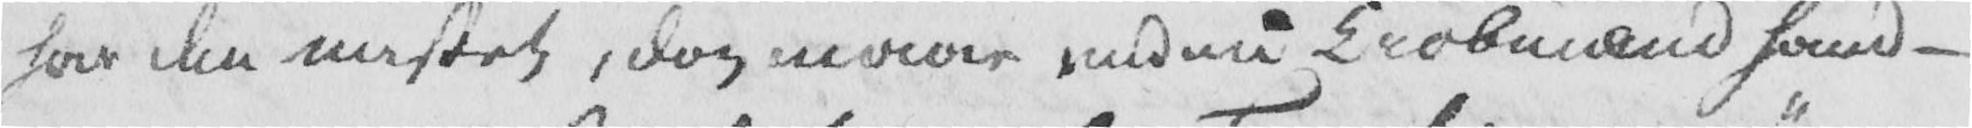har den mistet, dog maae endnu Kiøbmænd han-
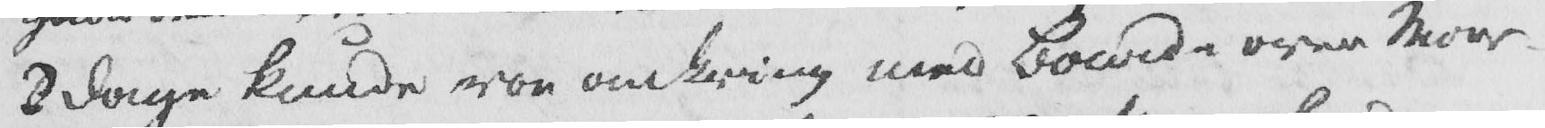2 Dage kunde roe omkring med Baade over Mar-
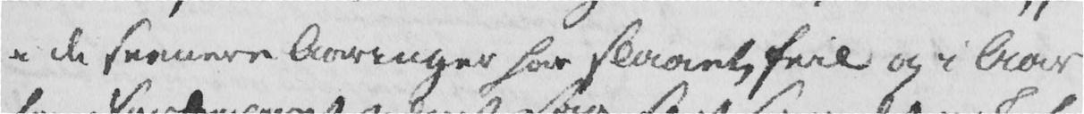i de seenere Aaringer har slaaet feil og i Aar
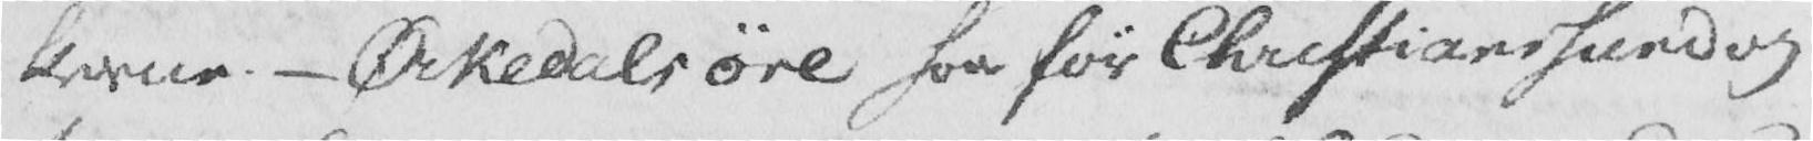kerne. - Ørkedalsøre har før Christianssund og
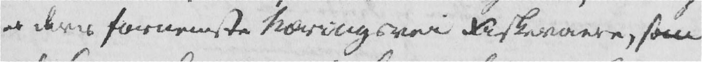er deres fornemste Næringsvei Fiskevarer, som
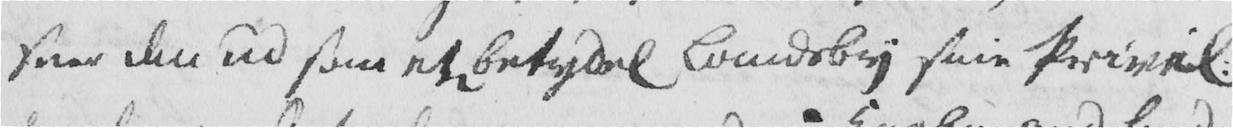seer den ud som et betydel Landsby sine Privil.
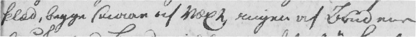klæd, begge smaae af Væxt, ingen af Brudene
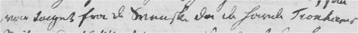var taget fra de Svenske da de havde Tronhiems
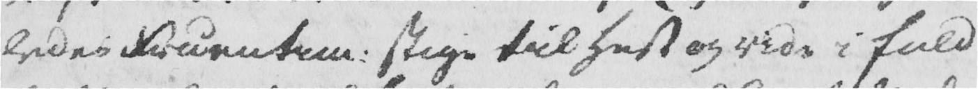ledes Fruentim. stige til Hest og ride i fuld
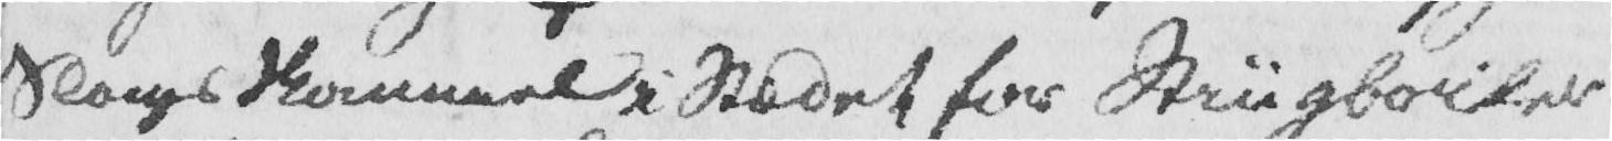Slags Skammel i stædet for Stiigbøiler
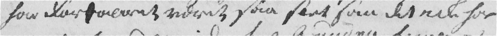har Foraaret været saa slet som det icke har
